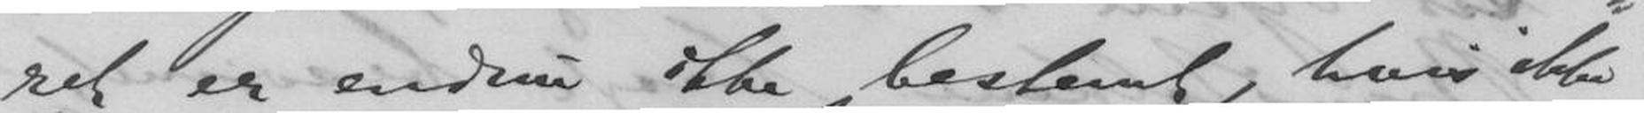ret er endnu ikke bestemt, hvis ikke
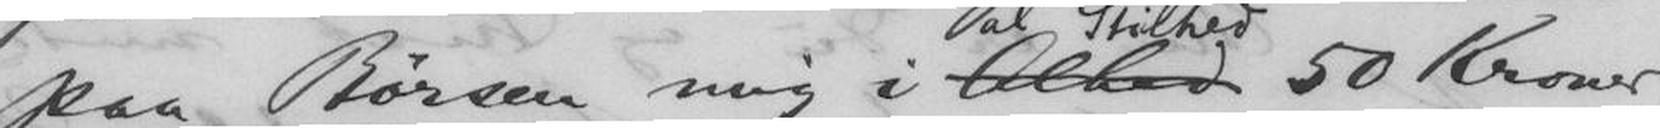paa Børsen mig i al Stilhed Alhed 50 Kroner
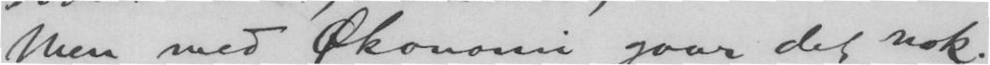- Men med Økonomi gaar det nok.
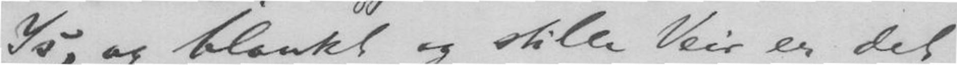Is, og blankt og stille Veir er det
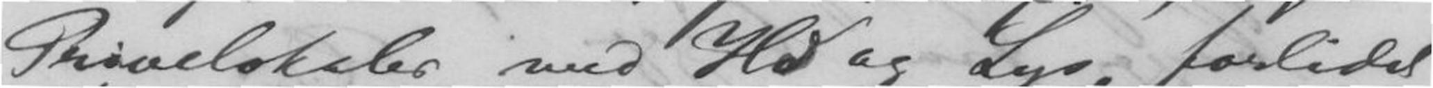Prøvelokaler med Ild og Lys, forlidet
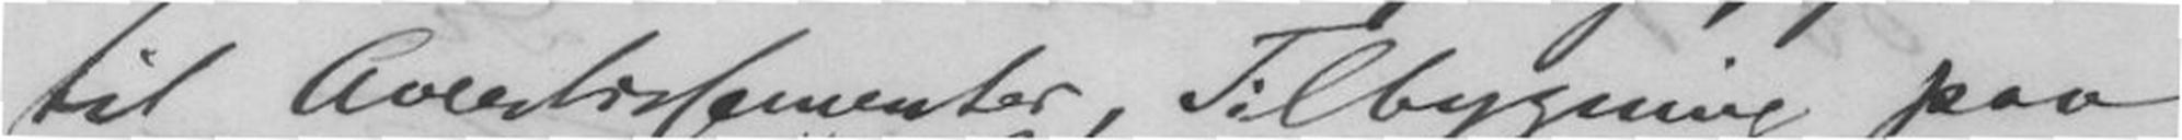til Avertissementer, Tilbygning paa
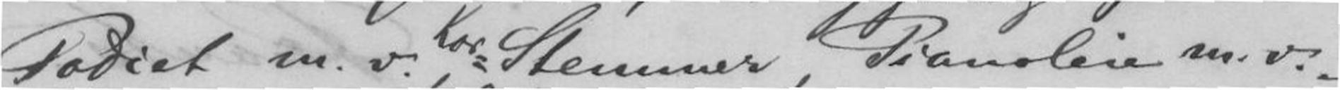Podiet m.v., Kor=Stemmer, Pianoleie m.v.
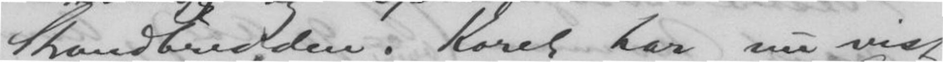Strandbredden. Koret har nu vist
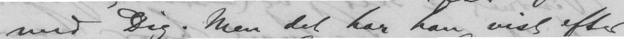med Dig. Men det har han vist efter
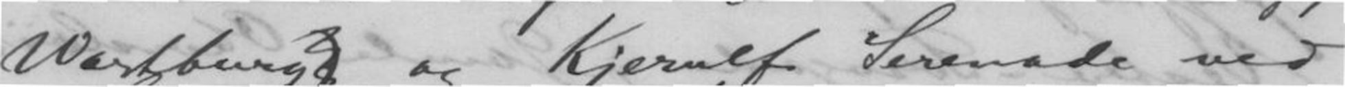Wartburg ) og Kjerulf Serenade ved
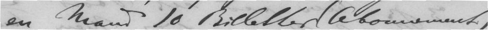en Mand 10 Biletter (Abonnement)
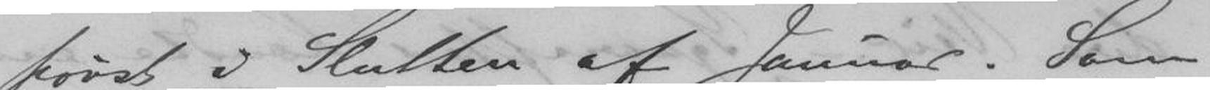først i Slutten af Januar. Som
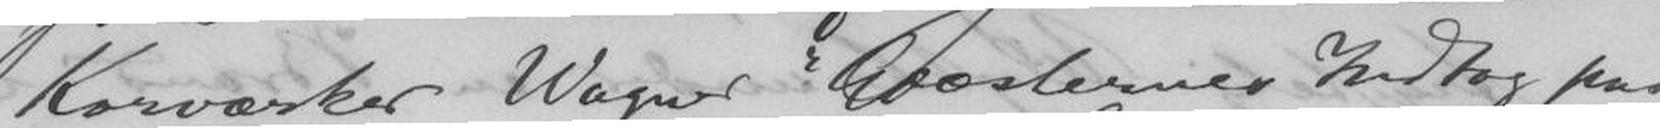Korværker Wagner "Gjæsternes Indtog paa
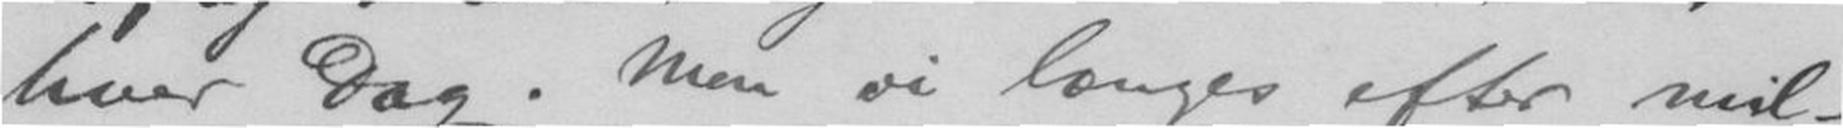hver Dag. Men vi længes efter mil-
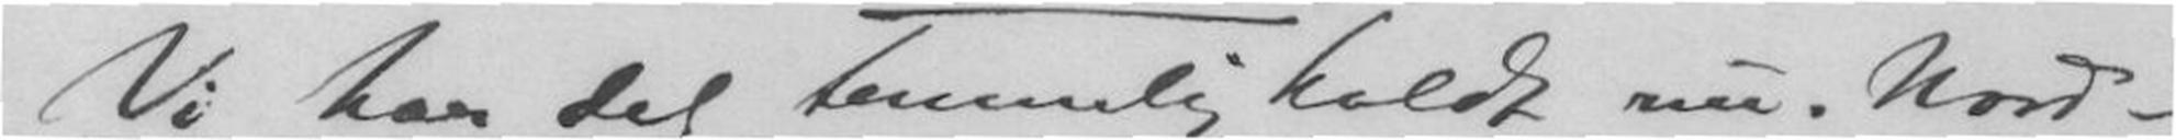Vi har det temmelig koldt nu. Nord-
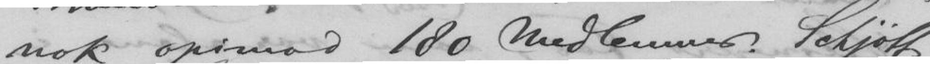nok opimod 180 Medlemmer. Schjøtt
